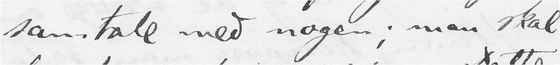samtale med nogen; man skal
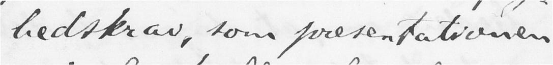hedskrav, som presentationen
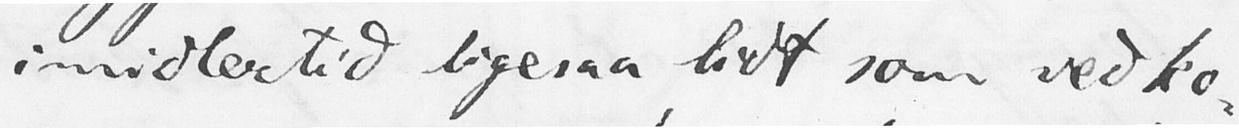imidlertid ligesaa lidt som ved ko-
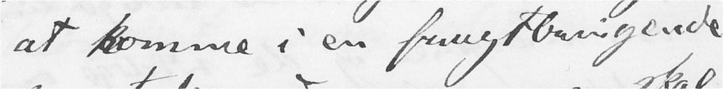at komme i en frugtbringende
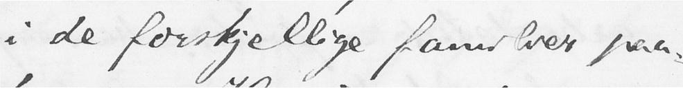i de forskjellige familier paa-
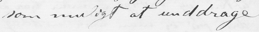som muligt at unddrage
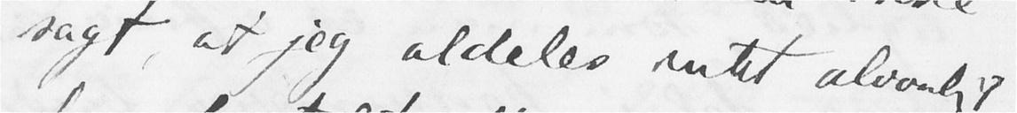sagt, at jeg aldeles intet alvorligt
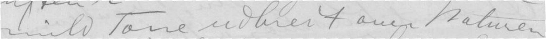mild Tone udbredt over Naturen,
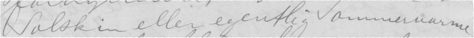Solskin eller egentlig Sommervarme.
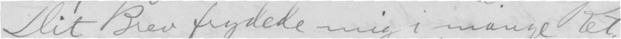Dit Brev frydede mig i mange Ret-
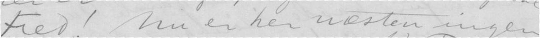Fred! Nu er her næsten ingen
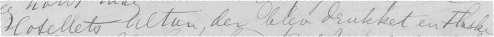Hotellets Altan, der blev drukket en Flaske
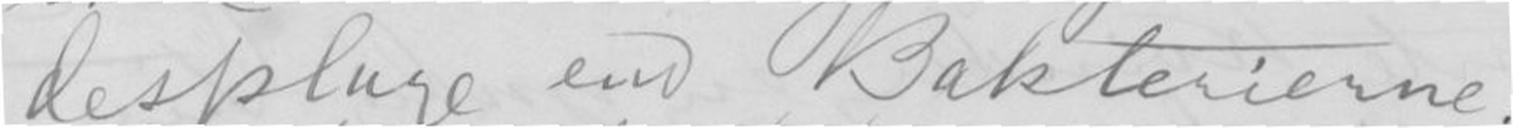desplage end Bakterierne.
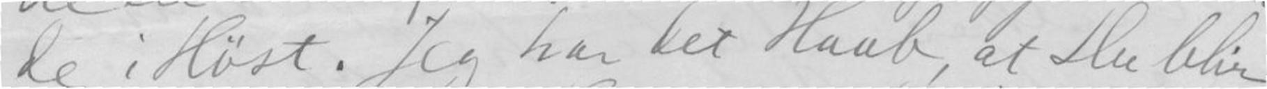de i Høst. Jeg har det Haab, at Du blir
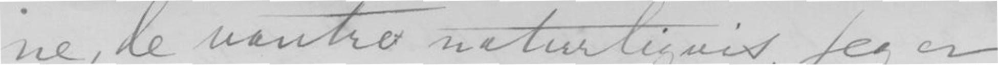ne, de vantro naturligvis. Jeg er
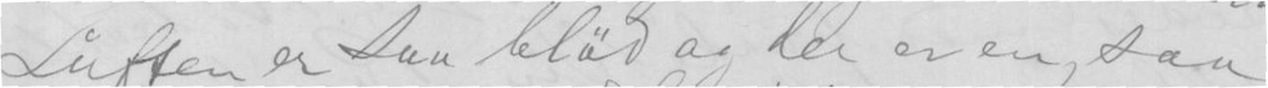Luften er saa blød og der er en saa
